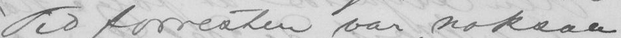Tid forresten var noksaa
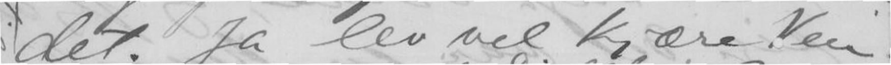det. Ja lev vel kjære Ven.
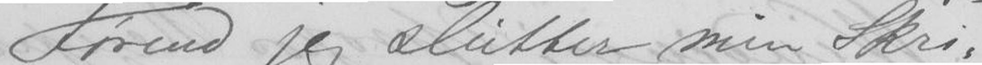Førend jeg slutter min Skri-
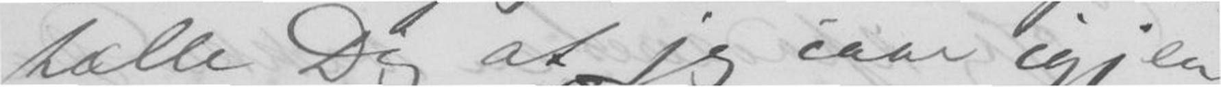tælle Dig at jeg iaar igjen
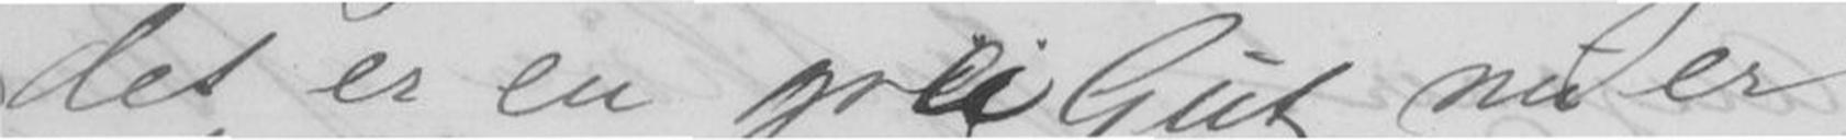det er en grei Gut nu er
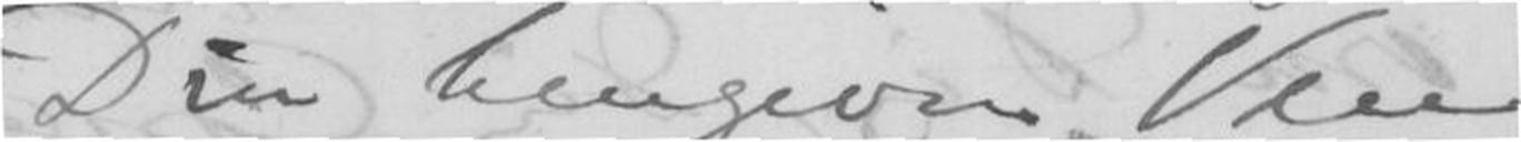Din hengivne Ven
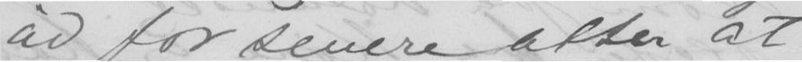ud for senere atter at
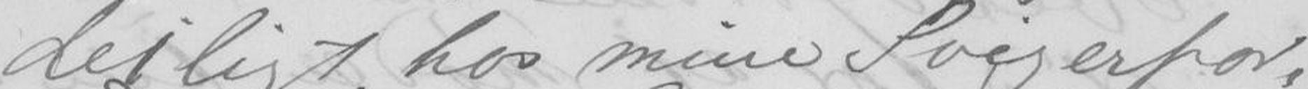deiligt hos mine Svigerfor-
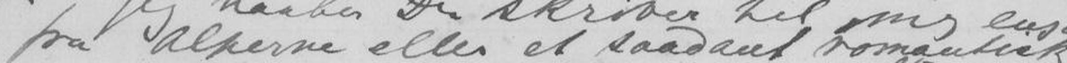fra Alperne eller at saadant romantisk
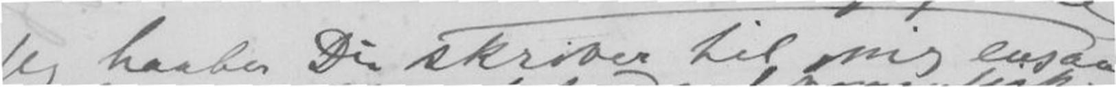Jeg haaber Du skriver til mig engang
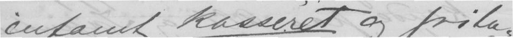infamt kasseret og frita-
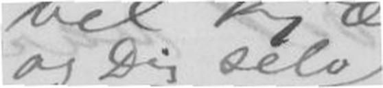Hils Din Kone mange Gange
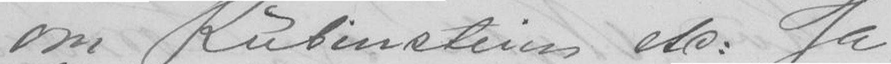om Rubinsteims etc.: Ja
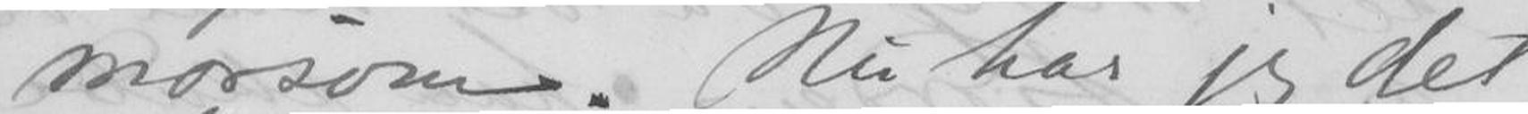morsom. Nu har jeg det
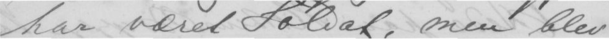har været Soliat, men blev
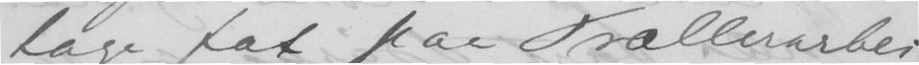tage fat paa Trallerarbei-
